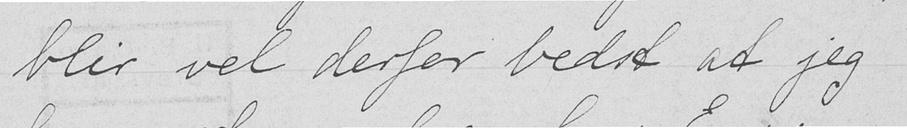blir vel derfor bedst at jeg
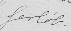forløb.
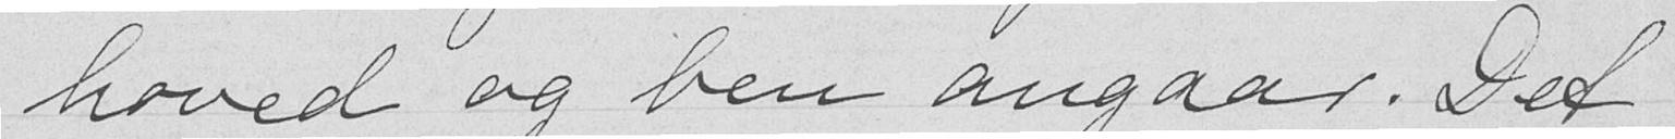hoved og ben angaar. Det
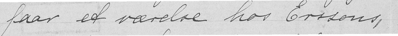faar et værelse hos Erssons,
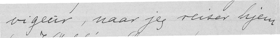vigeur, naar jeg reiser hjem
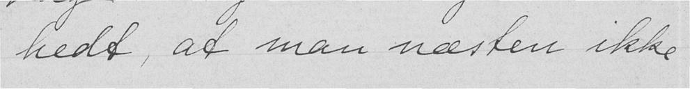hedt, at man næsten ikke
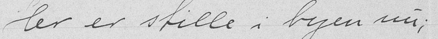Her er stille i byen nu;
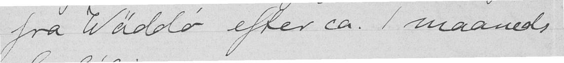fra Wäddø efter ca. 1maaneds
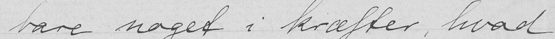bare noget i kræfter, hvad
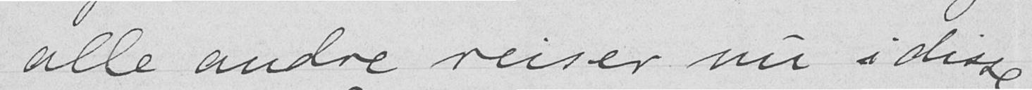alle andre reiser nu i disse
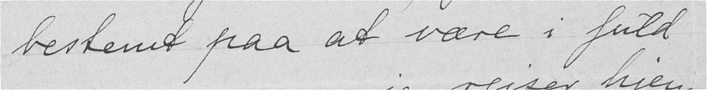bestemt paa at være i fuld
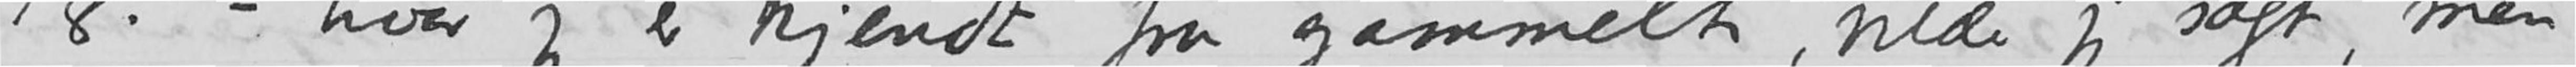/8. – hvor jeg er kjendt fra gammelt, vilde jeg sagt, men
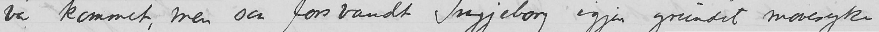var kommet, men saa forsvandt Ingjeborg igjen grundet mavesyke.
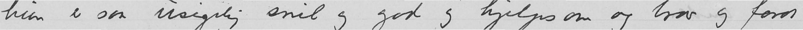hun er saa usigelig snil og god og hjelpsom og brav og fordi
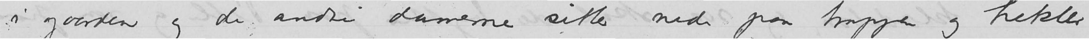i gaarden og de andre damerne sitter nede paa trappen og hekler
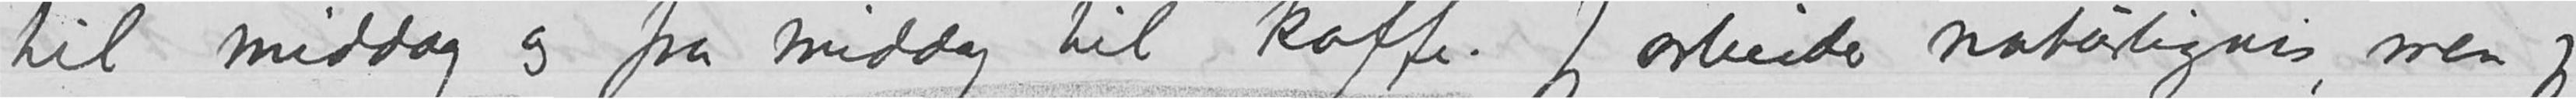til middag og fra middag til kaffe. Jeg arbeider naturligvis, men jeg
